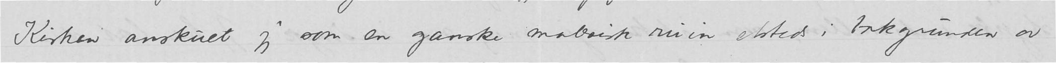Kirken anskuet jeg som en ganske malerisk ruin etsteds i bakgrunden av
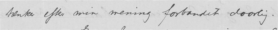tænker efter min, mening forbandet daarlig.
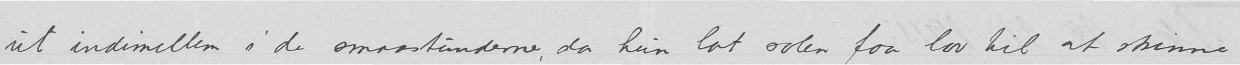ut indimellem i de smaastunderne, da hun lat solen faa lov til at skinne
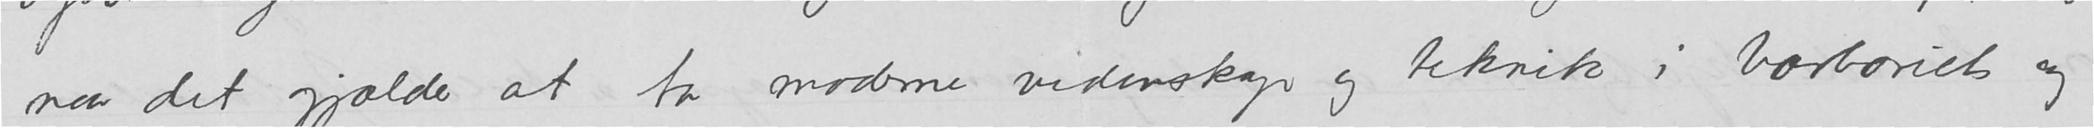naar det gjælder at ta moderne videnskap og teknik i barbariets og
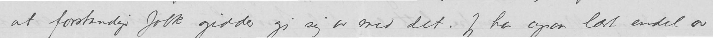at forstandige folk gidder gi sig av med det. Jeg har ogsaa læst en del av
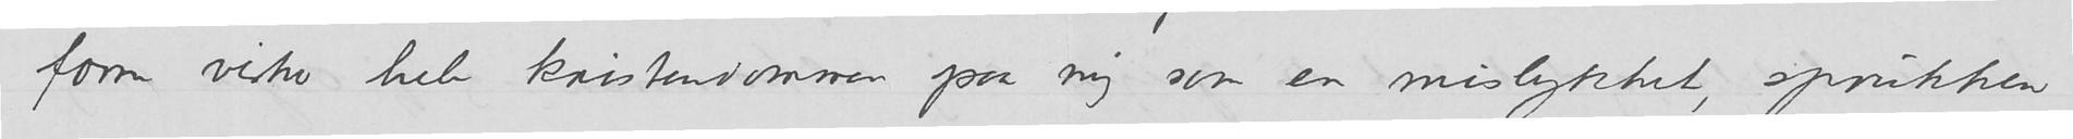form virker hele kristendommen paa mig som en mislykket, sprukken
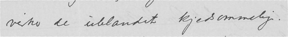virker de ublandet kjedsommelige.
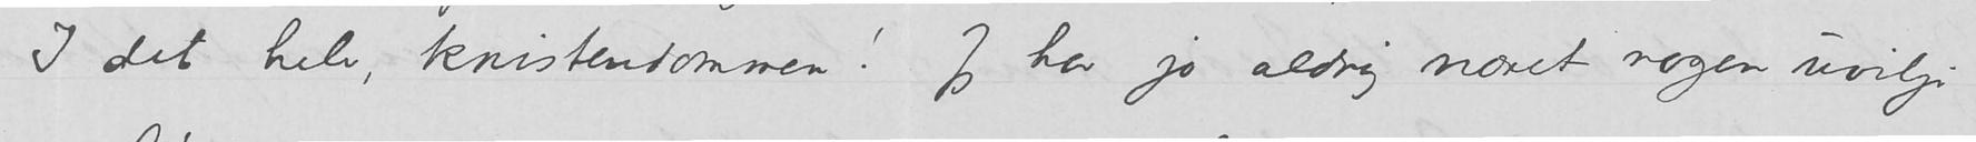I det hele, kristendommen! Jeg har jo aldrig næret nogen uvilje
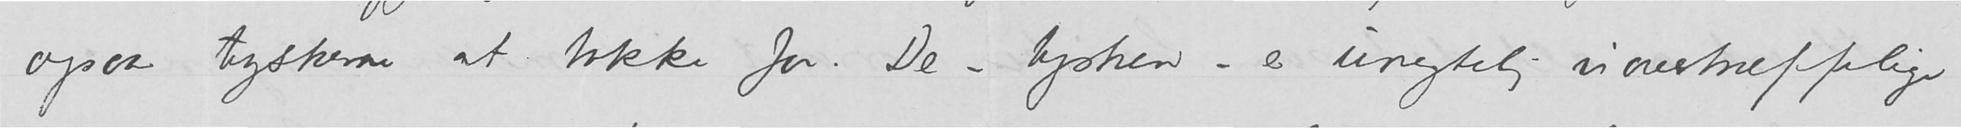ogsaa tyskerne at takke for. De – tyskere – er unegtelig uovertræffelige
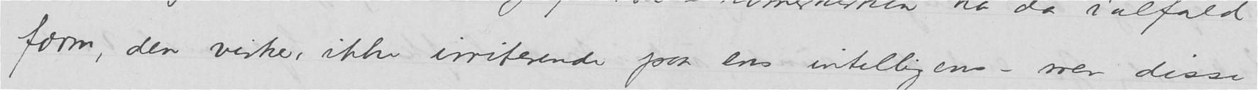form, den virker ikke irriterende paa ens intelligens – men disse
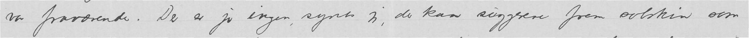var fraværende. Der er jo ingen, synes jeg, der kan suggerene frem solskinn som
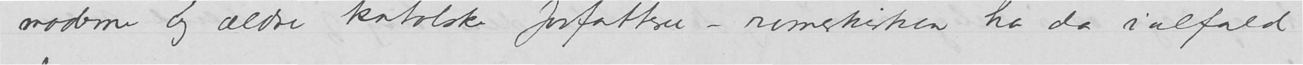moderne og ældre katolske forfattere – romerkirken har da ialfald
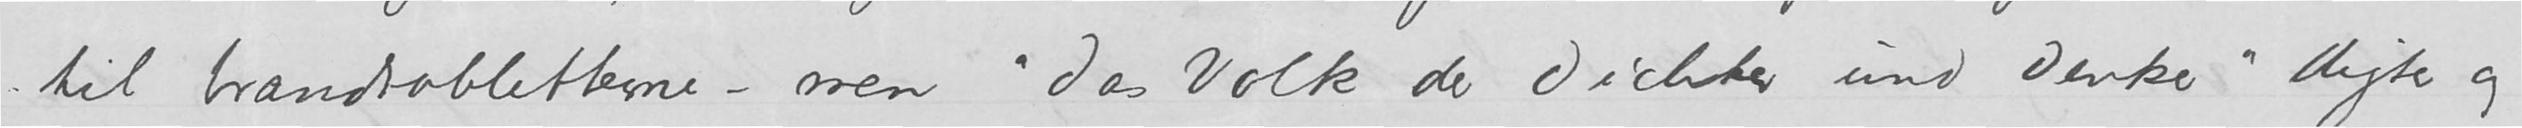til brandtabletterne – men «Das Volk der Dichter und Denker» digter og
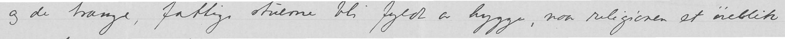og de trange, fattige stuerne bli fyldt av hygge, naar religionen et øieblikk
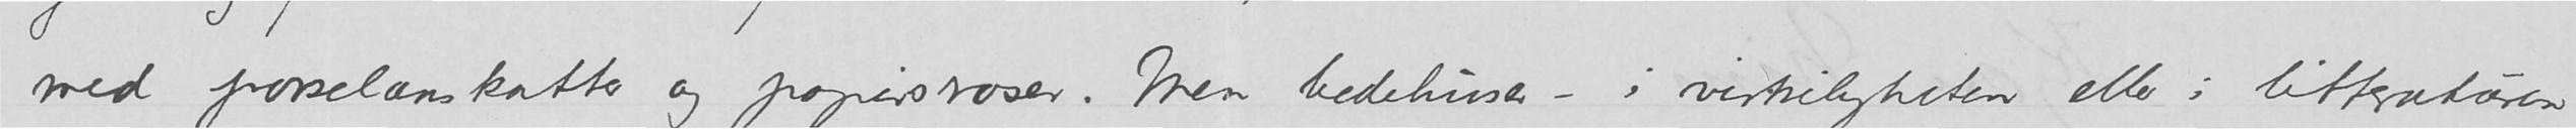med porselænskatter og papirsroser. Men bedehuser – i virkeligheten eller i litteraturen
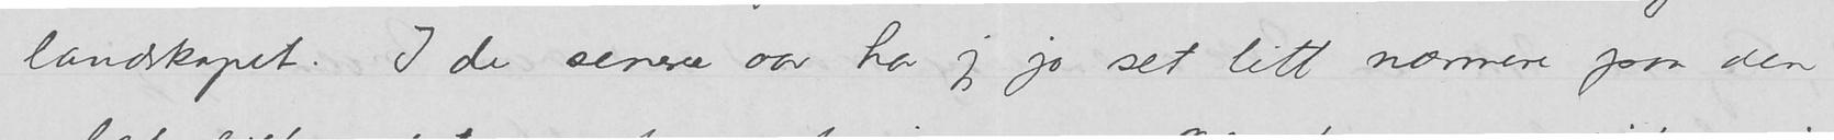landskapet. I de senere aar har jeg jo set litt nærmere paa den
